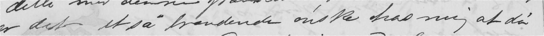er det et så brændende ønske hos mig at dø
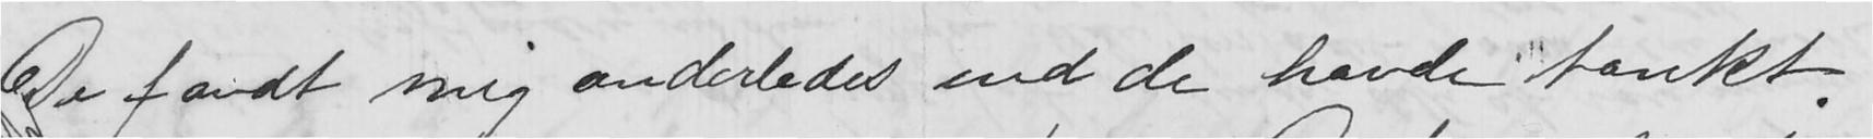De fandt mig anderledes end de havde tænkt.
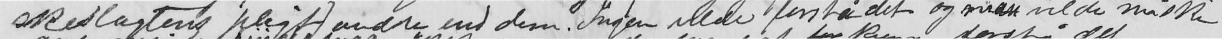skeslægtens pligt, andre end dem. Ingen vilde forstå det og man vilde måtte
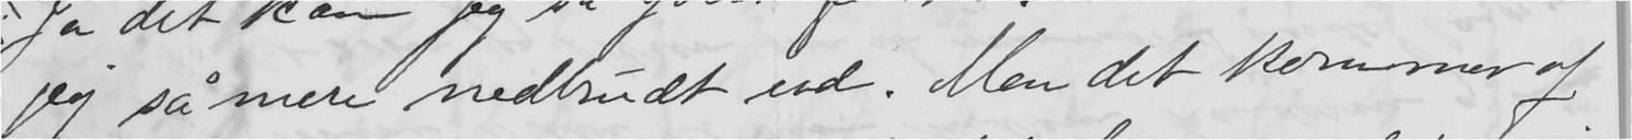jeg så mere nedbrudt ud. Men det kommer af
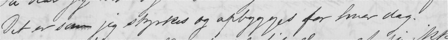Det er som jeg styrkes og opbygges for hver dag!
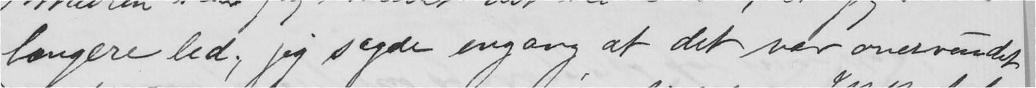længre led, jeg sagde engang at det var overvundet
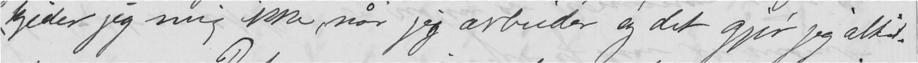kjeder jeg mig ikke, når jeg arbeider og det gjør jeg altid.
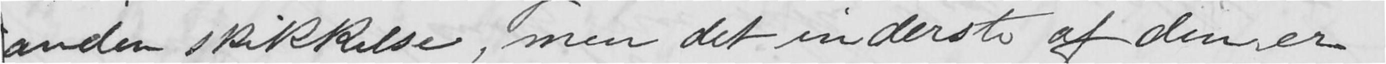anden skikkelse, men det inderste af den er
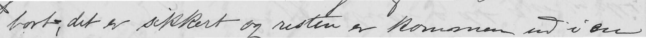bort, det er sikkert og resten er kommen ud i en
